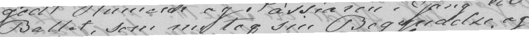Ballet, som nu tog sin Begyndelse, og
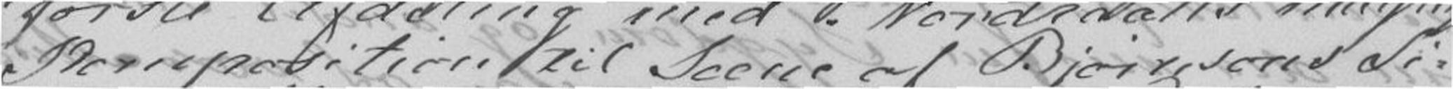Komposition til Scene af Bjørnsons Si-
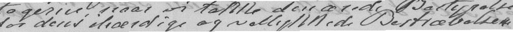for dens ihærdige og vellykkede Bestræbelse
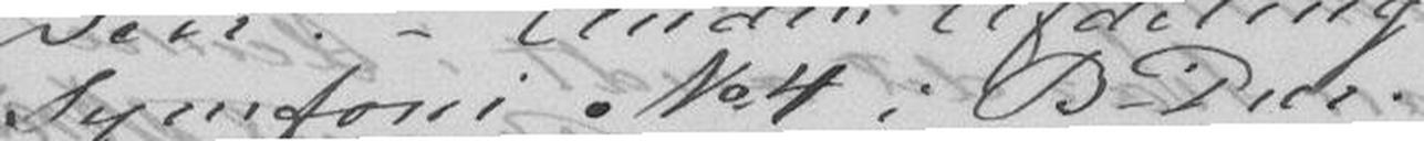Sumfoni N. 4 i B-Dur.
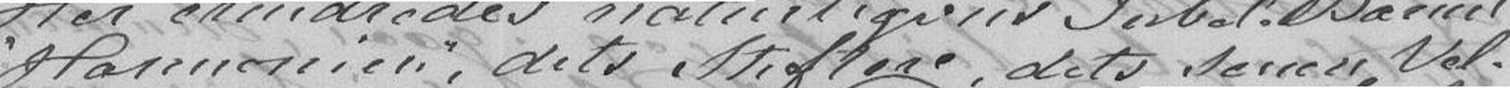"Harmonien", dets Stiftere, dets senere Vel-
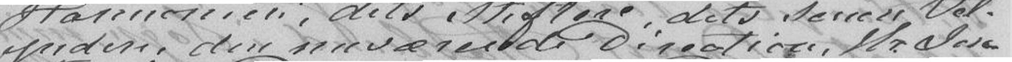yndere, den nuværende Direction, Hr. In-
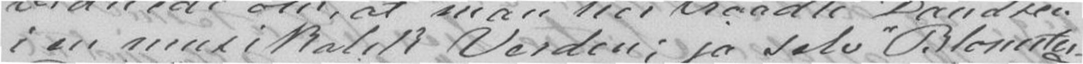i en musikalsk Verden; ja selv "Blomster-
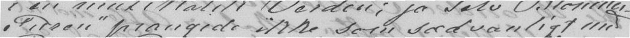prisen" prangede ikke som sædvanligt med
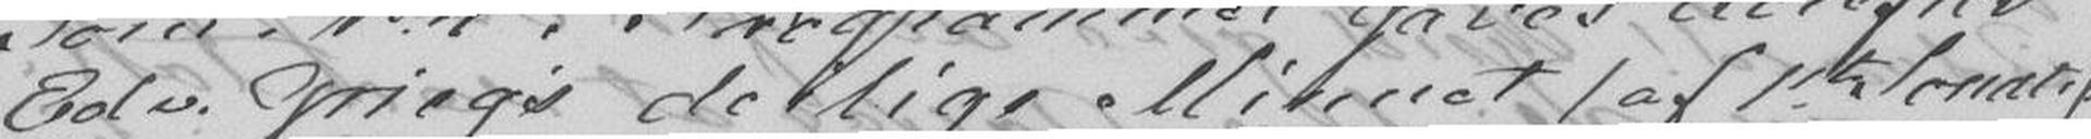Edv. Griegs deilige Minnet (af 1ste Sonate)
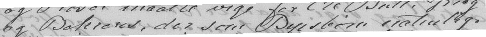og Behrens, der som Byesbørn naturlig-
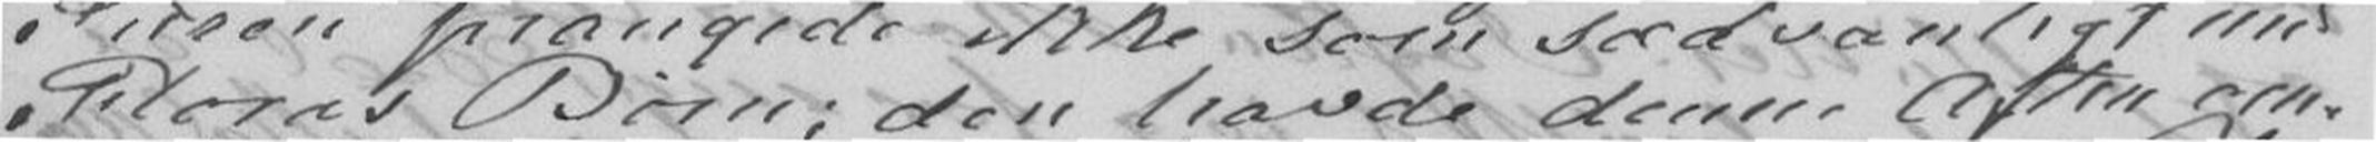Floras Børn; den havde denne Aften om-
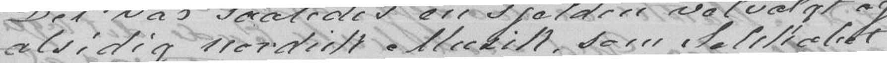alsidig nordisk Musik, som Selskabet
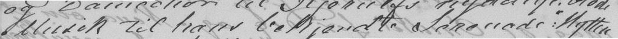Musik til hans bekjendte Serenade "Hytten
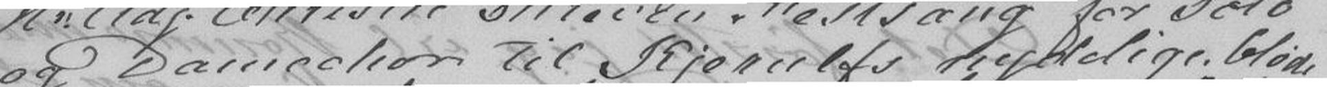og Damechor til Kjerulf nydelige bløde
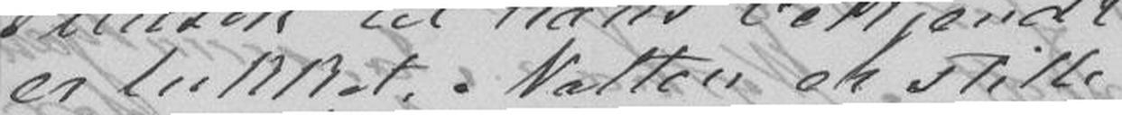er lukket, Natten er stille"
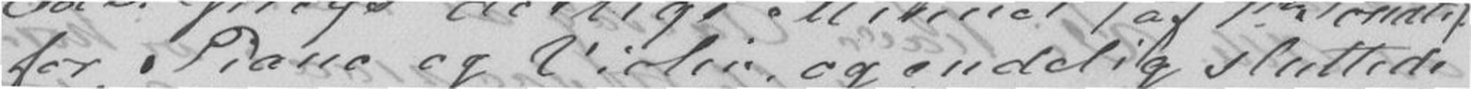for Piano og Violin, og endelig Sluttede
